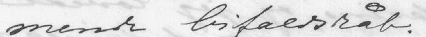mende bifaldsråb.-
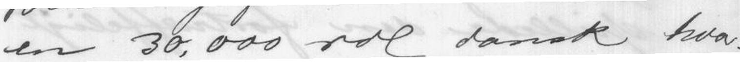en 30, 000 rdl dansk hvor-
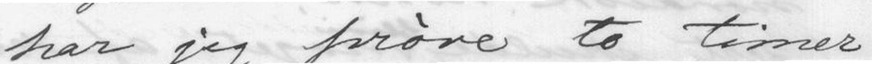har jeg prøve to timer
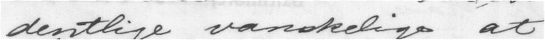dentlige vanskelige at
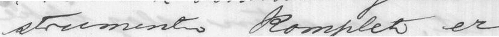strumenter Komplet er
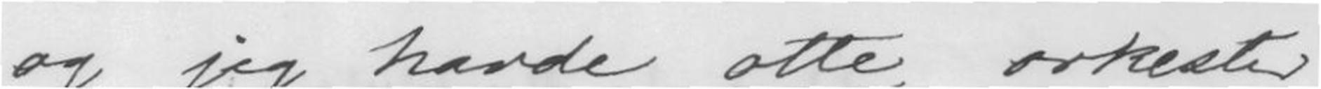og jeg havde otte orkester-
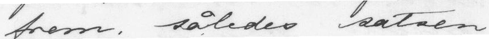frem. Således satsen
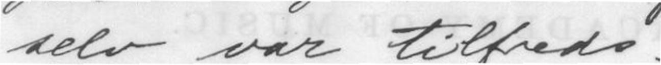selv var tilfreds.
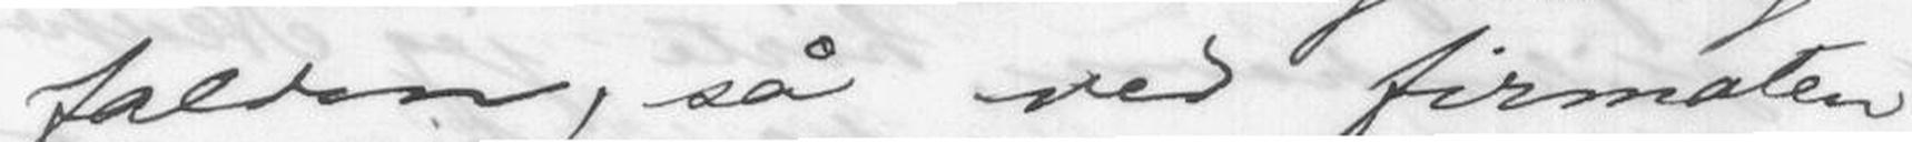faldent, så ved firmaten
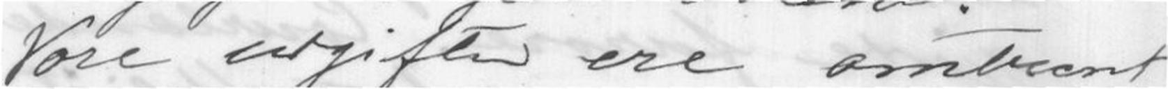Vore avgifter ere omtrent
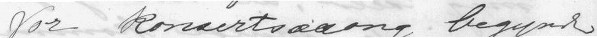Vor Konsertsæsong begynte
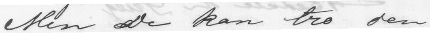Men Du kan tro den
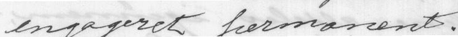engageret permanent.
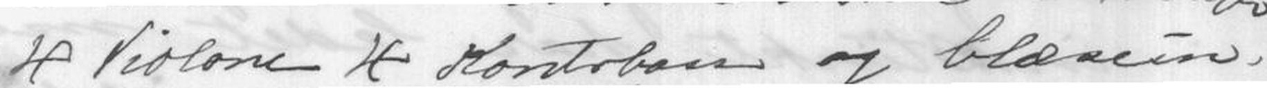4 Violone 4 Kontrbass og blæsein-
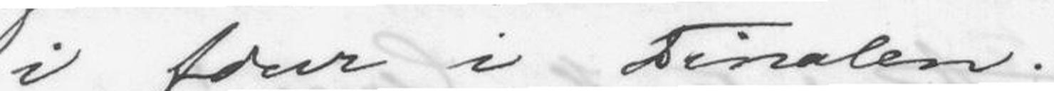i fører i Finalen.
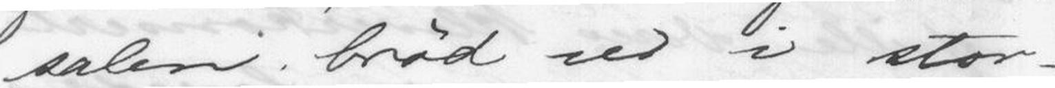salen brød ud i stor-
